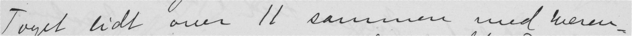Toget lidt over 11 sammen med Weren-
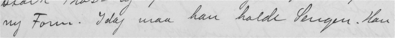ny Form. Idag maa han holde Sengen. Han
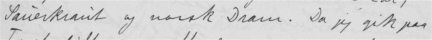Sauerkraut og norsk Dram. Da jeg gik paa
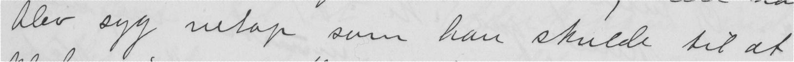blev syg netop som han skulde til at
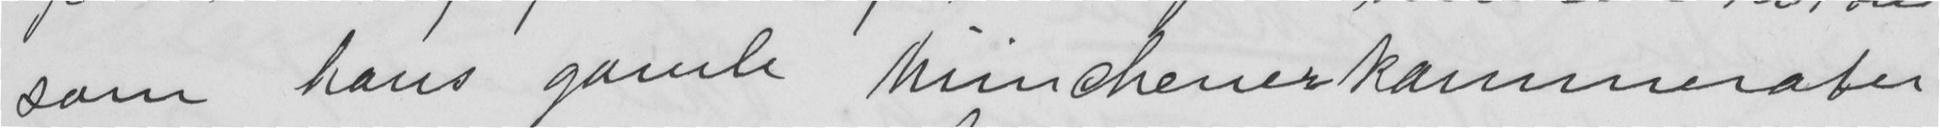som hans gamle Müchenerkammerater
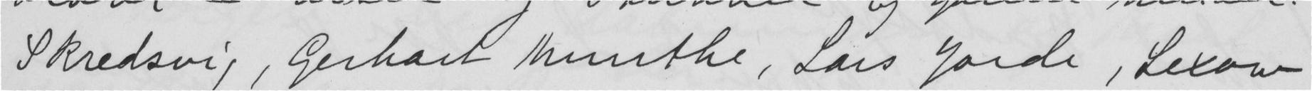Skredsvig, Gerbart Munthe, Lars Jorde, Lexow
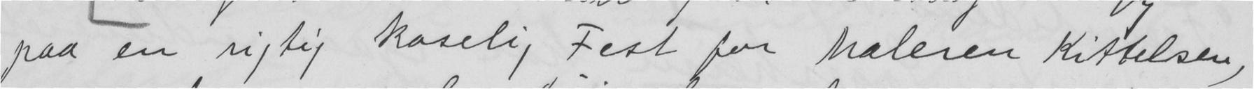paa en rigtig koselig Fest for Maleren Kittelsen,
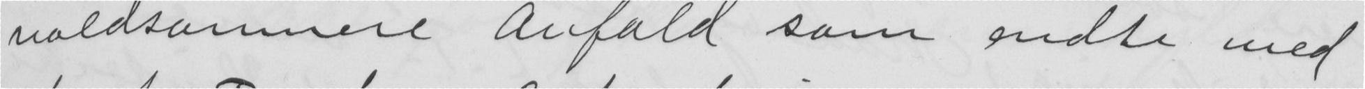voldsomnere Anfald som endte med
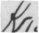Kr.
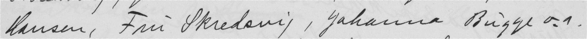Hansen, Fru Skredsvig, Johanna Bugge o.a.
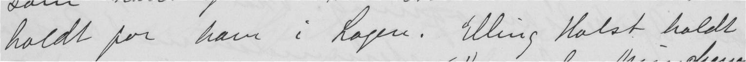holdt for ham i Logen. Elling Holst holdt
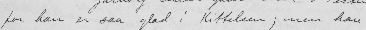for han er saa glad i Kittelsen; men han
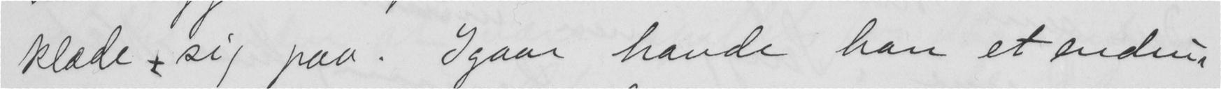klæde sig paa. Igaar havde han et endnu
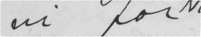er for
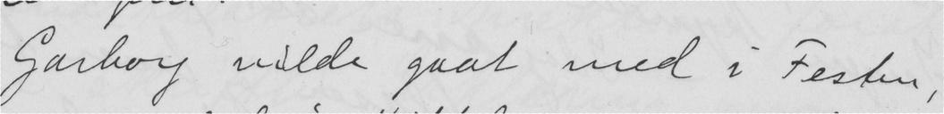Garborg vilde gaat med i Festen,
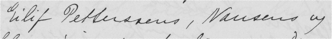Eilif Petterssens, Nansens og
